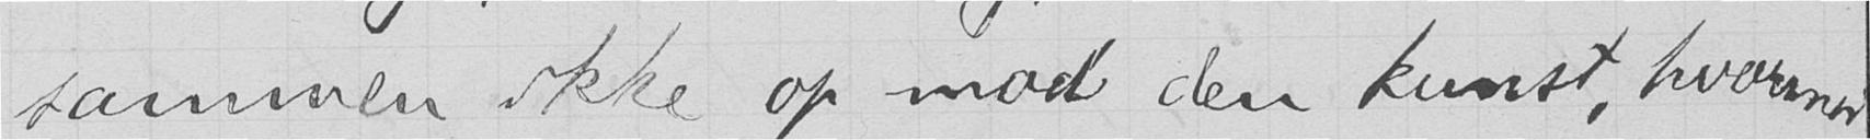sammen ikke op mod den kunst, hvormed
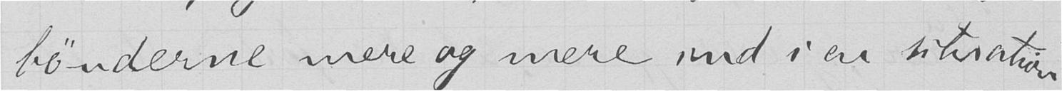bønderne mere og mere ind i en situation,
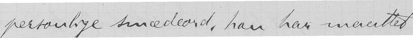personlige smædeord, han har maattet
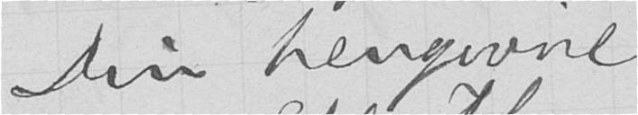Din hengivne
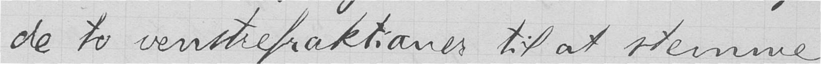de to venstrefraktioner til at stemme
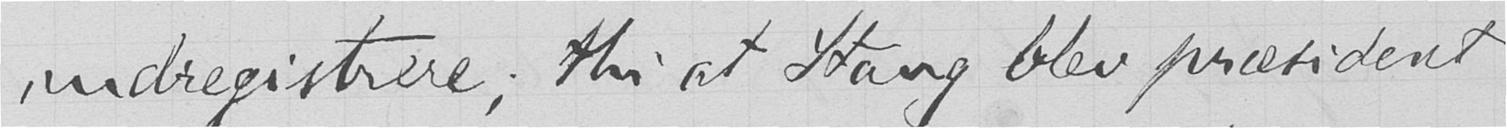indregistrere; thi at Stang blev præsident
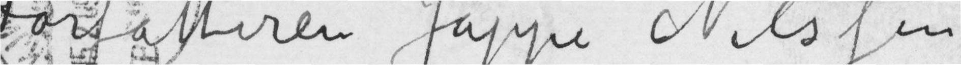Forfatteren Jappe Nilssen
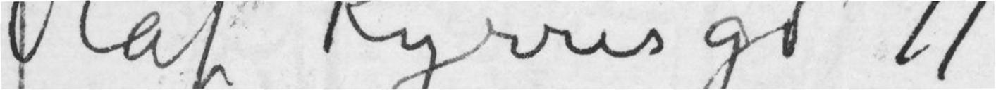Olaf Kyrres gd 11
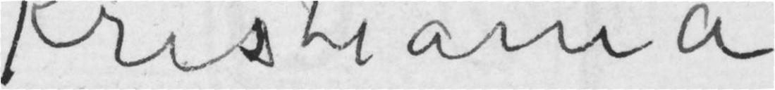Kristiania
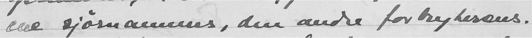ene sjømannens, den andre forbryterens.
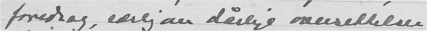foredrag, særlig om dårlige oversettelser -
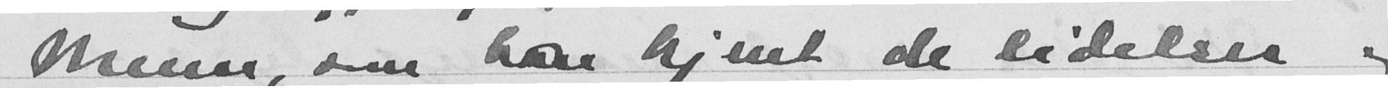Menn, som har kjent de lidelser og
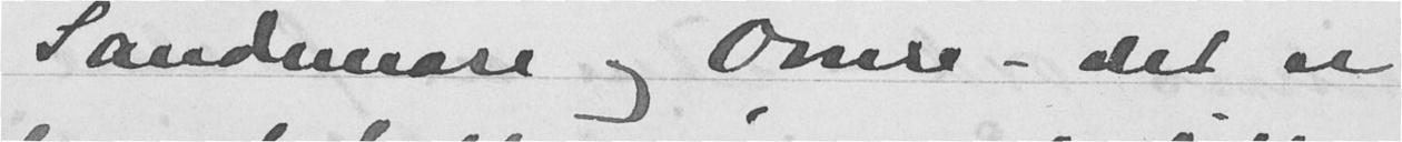Sandemose og Omre - det er
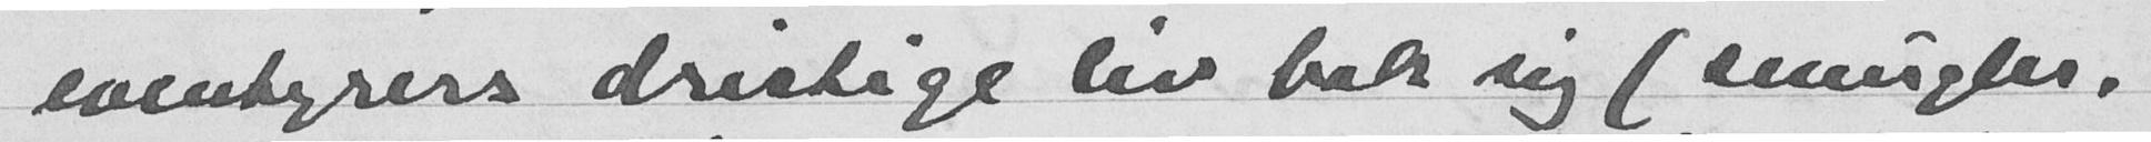eventyrers dristige liv bak sig (smugler,
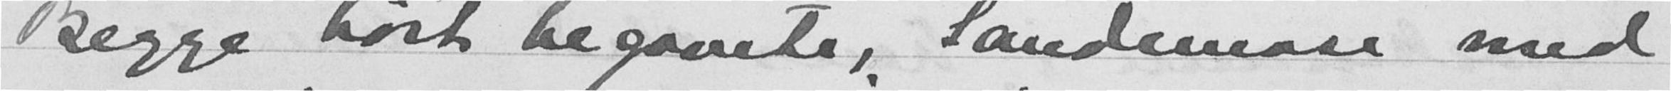Begge høit begavete, Sandemose med
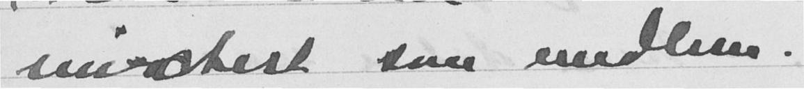invitert som medlem.
